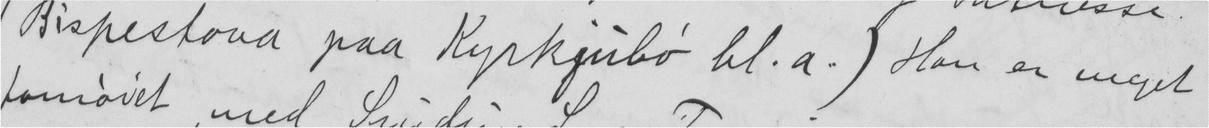(Bispestova paa Kyrkjubø bl.a.) Han er meget
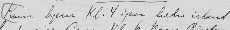Kom hjem Kl. 4 igaar bedre istand
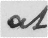at
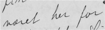været her for
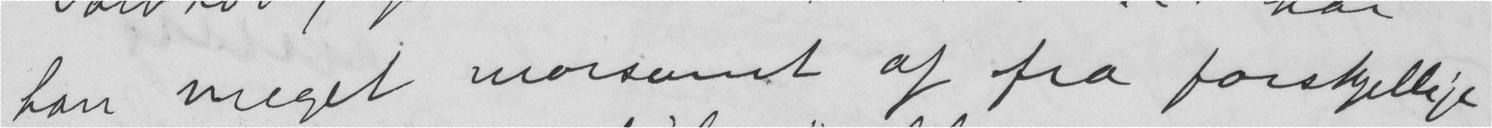han meget morsomt af fra forskjellige
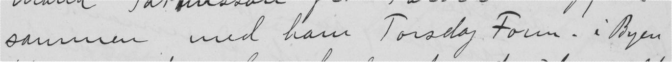sammen med ham Torsdag Form. i Byen
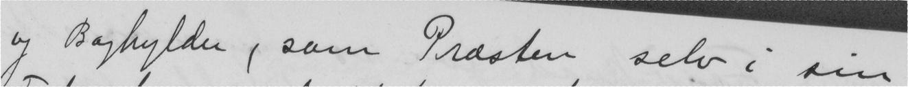og Boghylder, som Præsten selv i sin
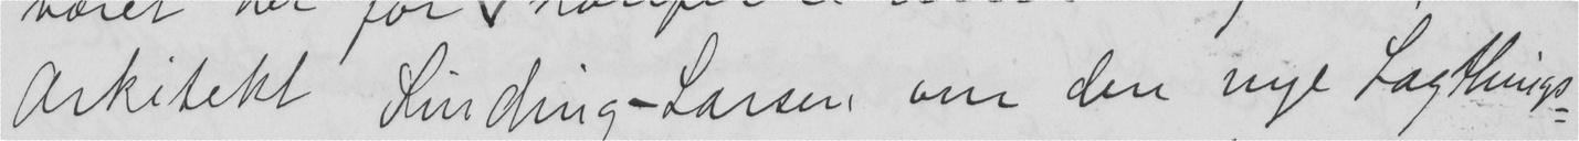Arkitekt Sinding-Larsen om den nye Lagthings-
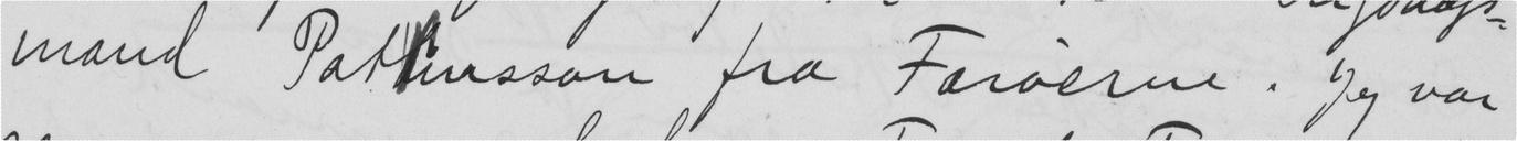mand Patiusson fra Færøerne. Jeg var
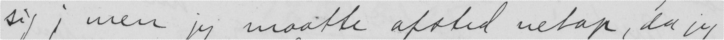sig; men jeg maatte afsted netop, da jeg
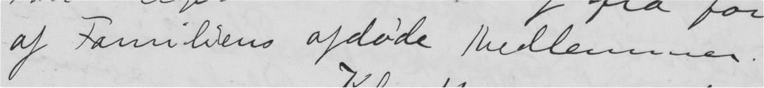af Familiens afdøde Medlemmer.
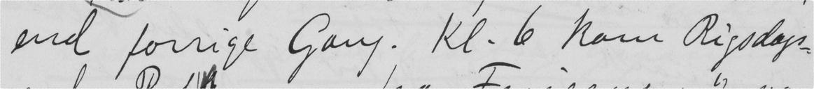end forrige Gang. Kl. 6 kom Rigsdags-
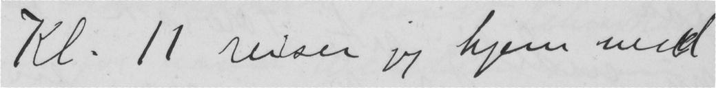Kl. 11 reiser jeg hjem med
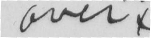over
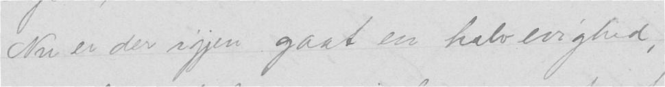Nu er der igjen gaat en halv evighed,
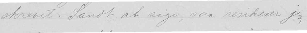skrevet. Sandt at sige, saa resikerer jeg
